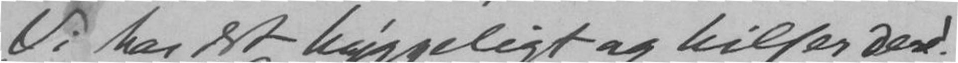Vi har det hyggeligt og hilser dere!
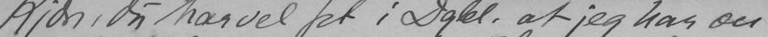Kjære, du har vel set i Dgbl. at jeg har æn-
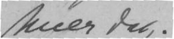hver dag.
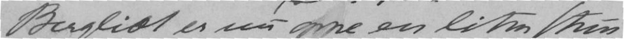Bergliot er nu oppe en liden stun
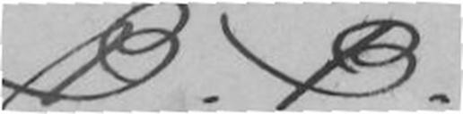B. B.
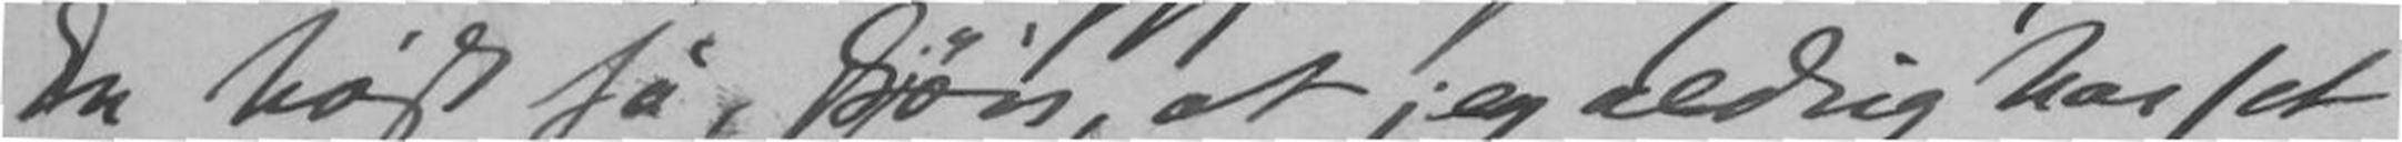En høst så skjøn, at jeg aldrig har set
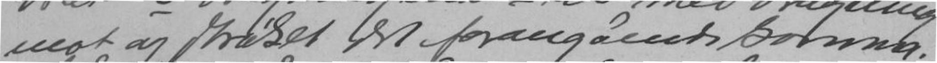mot og strøket det forangående komma.
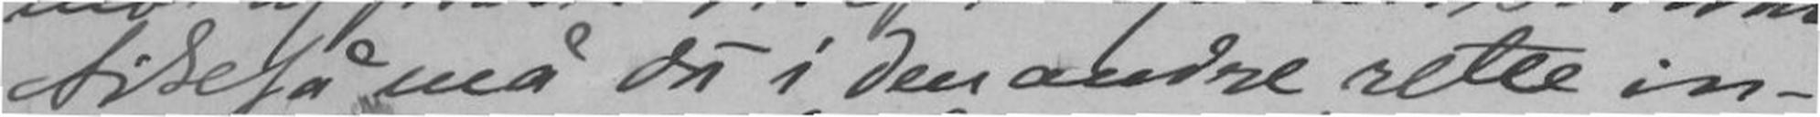Likeså må du i den andre rette in-
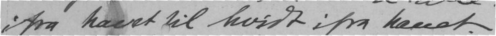ifra havet til hvidt ifra havet.
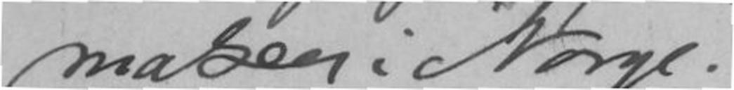maken i Norge.
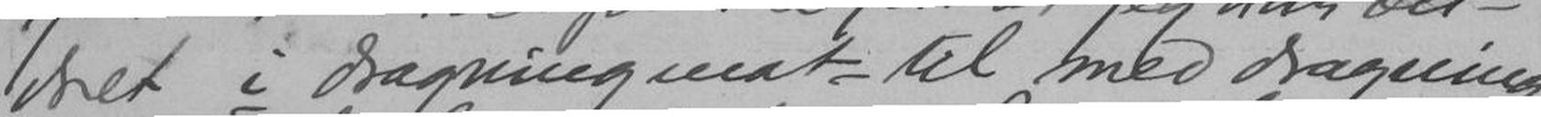dret i dragning mot til med dragning
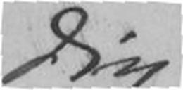Din
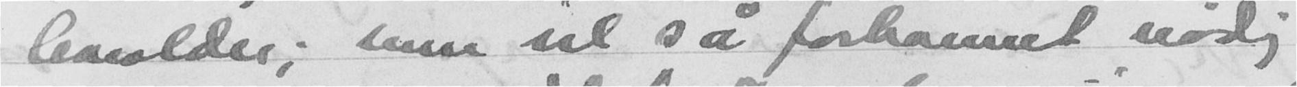senalder; men vil så forbannet nødig
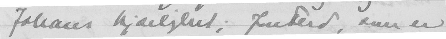Johans kjærlighet; fankeid, som er
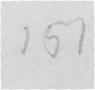157
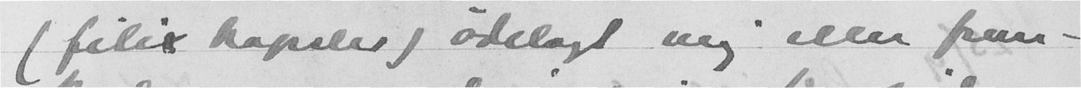(Felix kapsler) ødelagt mig eller frem-
